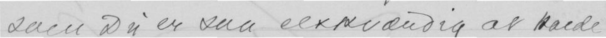som Du er saa elskværdig at kalde
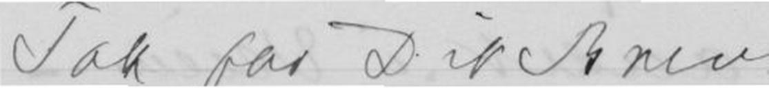Tak for Dit Brev
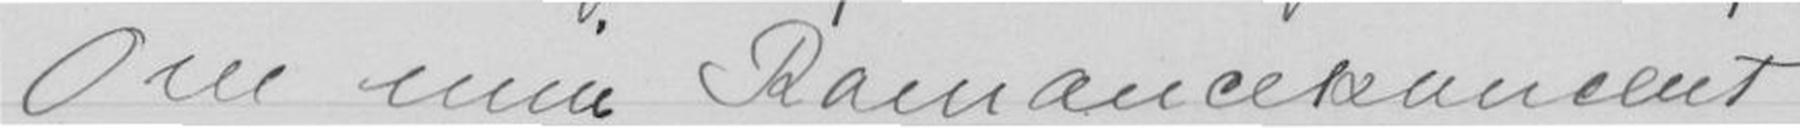Om min Romancekoncert
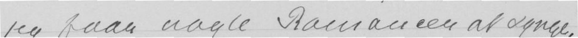jeg faar nogle Romancer at synge
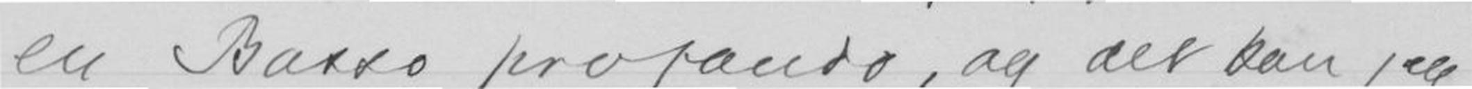en Basso propando, og det kan jeg
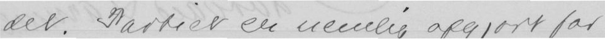det. Partiet er nemlig opgjort for
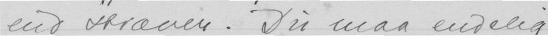end stræver. Du maa endelig
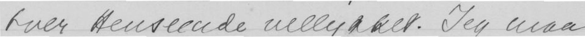hver henseende vellykket. Jeg maa
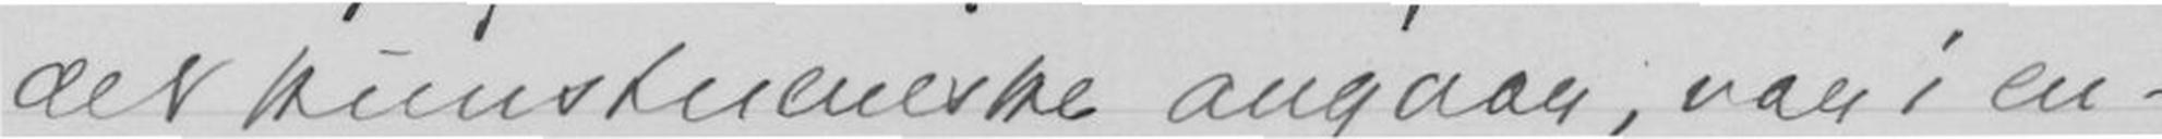det kunstneriske angaar, var i en-
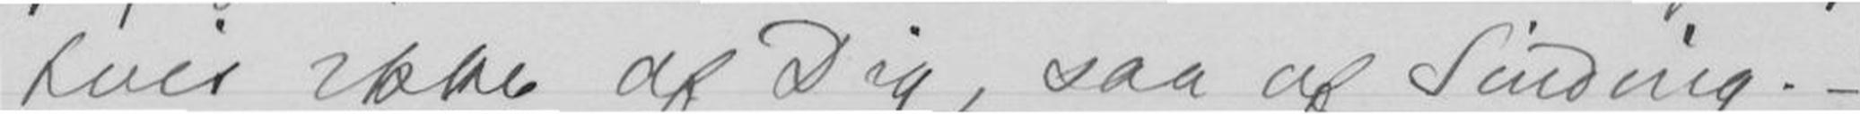hvis ikke af Dig, saa af Sindnig. -
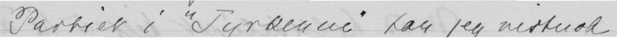Partiet i "Tyrkerne" kan jeg vistnok
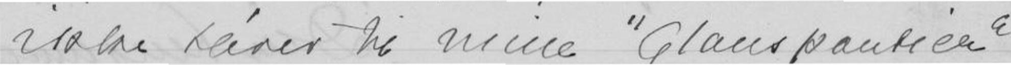ikke hærer til mine "Glanspartier"
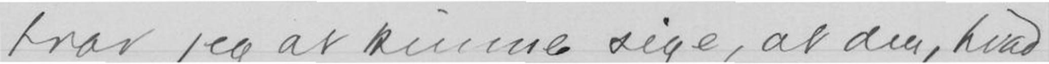tror jeg at kunne sige, at den, hvad
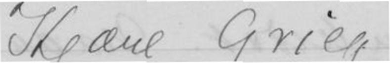Kjære Grieg
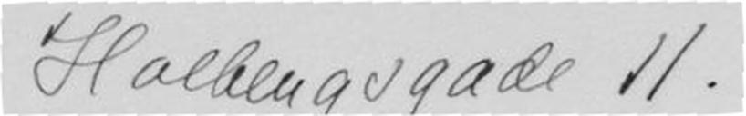Holbergsgade 11
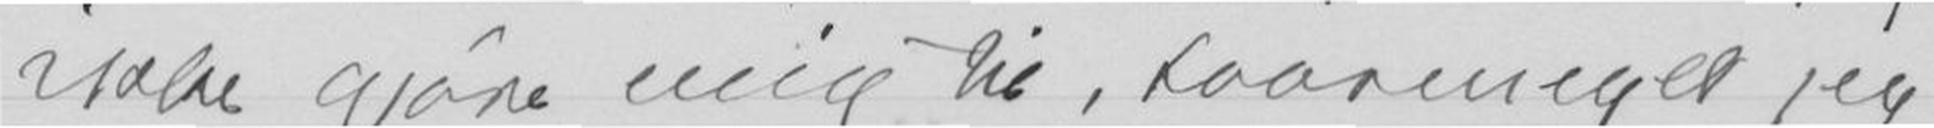ikke gjøre mig til, saanumeget jeg
